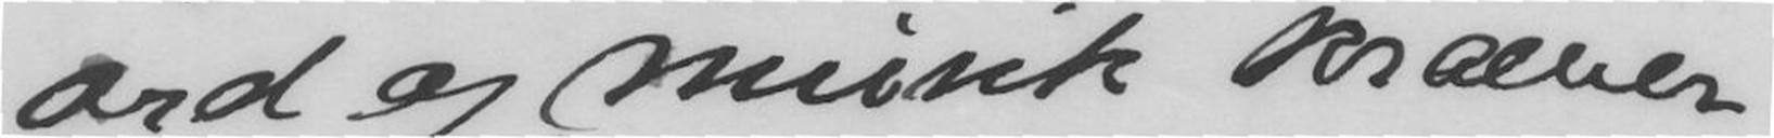ord og musik kræver.
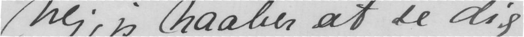Nej, jeg haaber at se dig
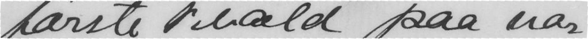første kvæld paa vor
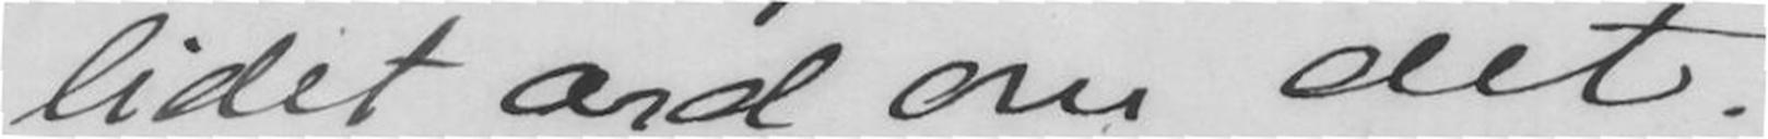lidet ord om det.
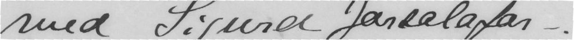med Sigurd Jorsalfar -.
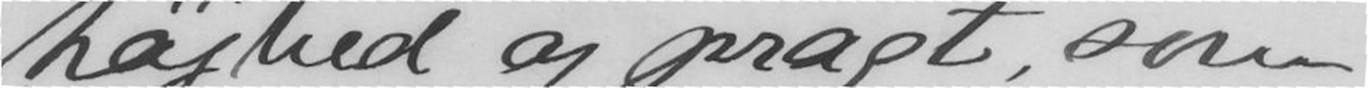højhed og pragt, som
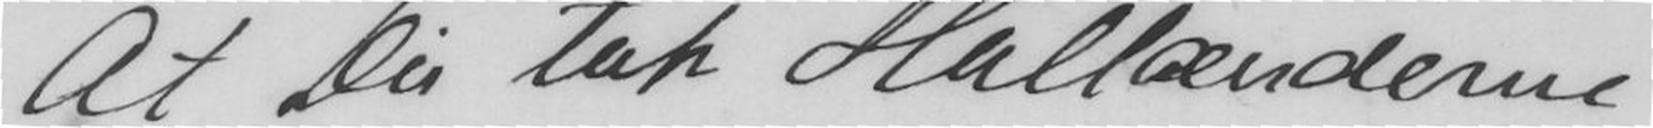At du tok Hollænderne
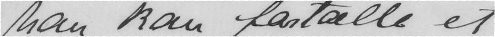han kan fortælle et
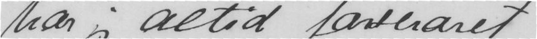har jeg altid forsvaret -,
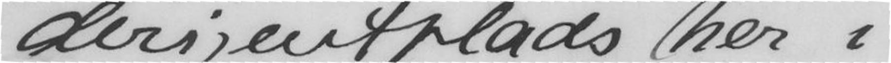dirigentplads her i
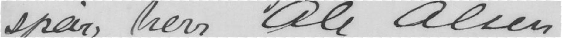spørg herr Ole Olsen,
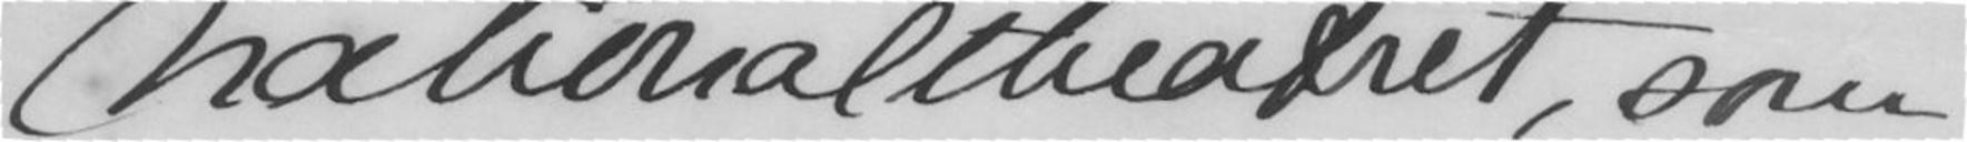Nationaltheatret, som
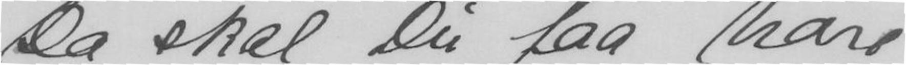Da skal du faa høre
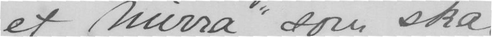et hurra som skal
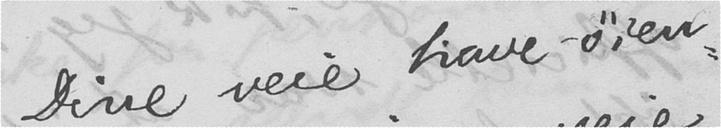Dine veie have øien-
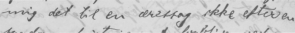mig det til en æressag ikke efter en
